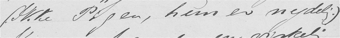(Ikke Pigen, hun er nydelig.)
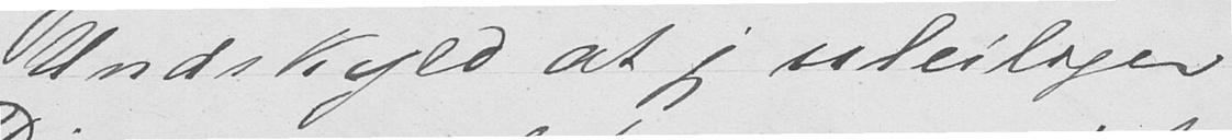Undskyld at jeg uleiliger
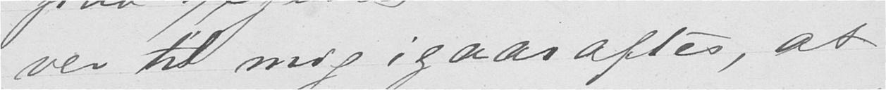ver til mig igaaraftes, at
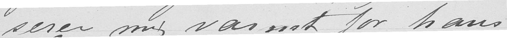serer mig varmt for hans
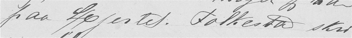paa Hjertet. Folkestad skri-
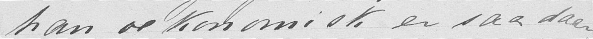han oekonomisk er saa daar-
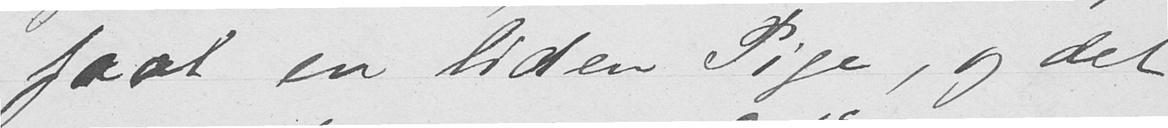faat en liden Pige, og det
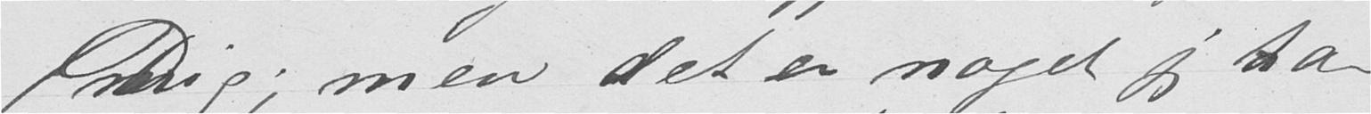Mig; men det er noget jeg har
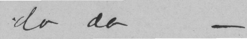do do -
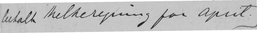betalt Melkeregning for April.
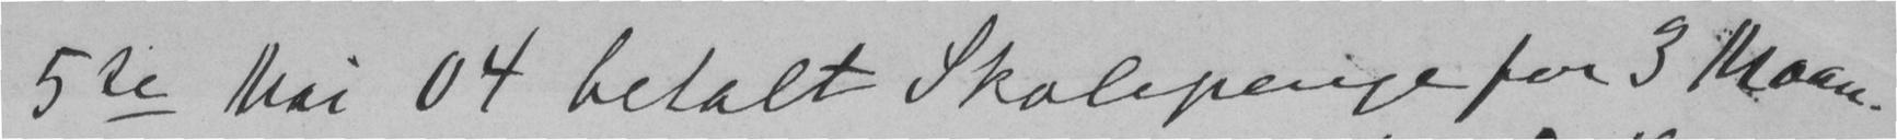5te Mai 04 betalt Skolepenge for 3 Maan.
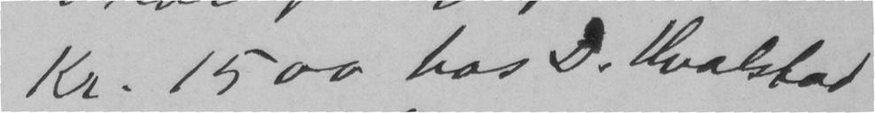Kr. 1500 hos D. Hvalstad
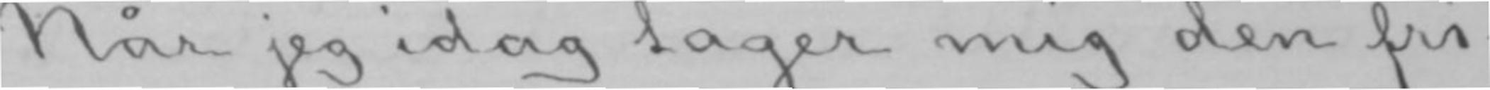Når jeg idag tager mig den fri-
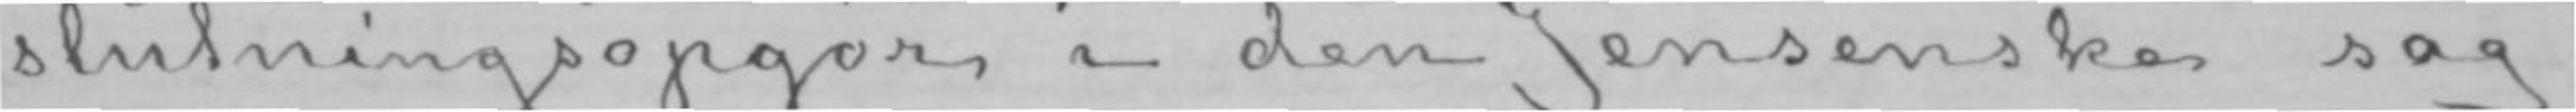slutningsopgør i den Jensenske sag.
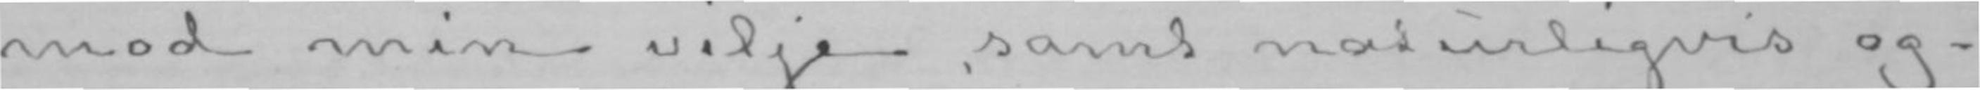mod min vilje, samt naturligvis og-
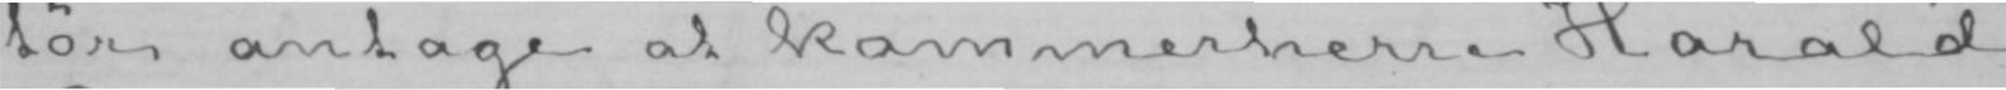tør antage at kammerherre Harald
